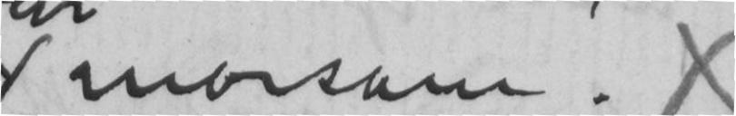morsom. x
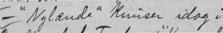- "Nylænde" knuser idag i
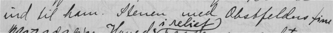ind til ham. Stenen med Obstfelders fine
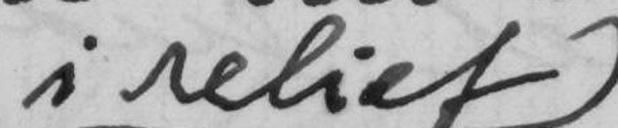i relief
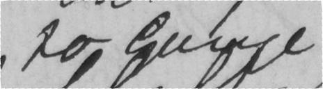to Gange
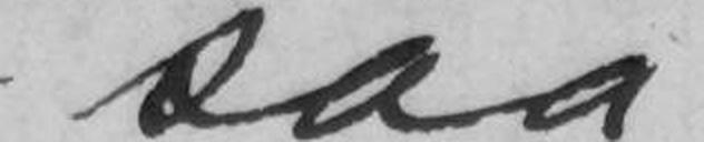saa
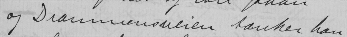og Drammensveien tænker han
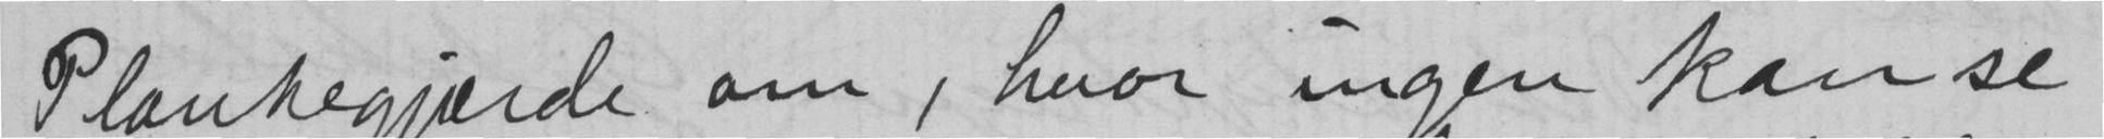Plankegjærde om, hvor ingen kan se
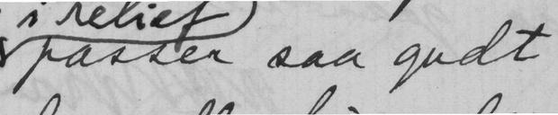passer saa godt
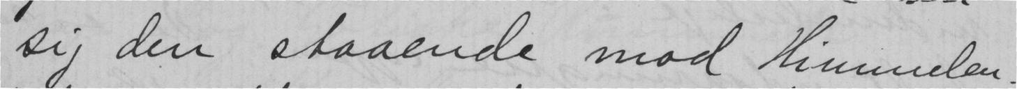sig den staaende mod Himmelen.
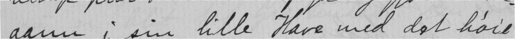aann i sin lille Have med det høie
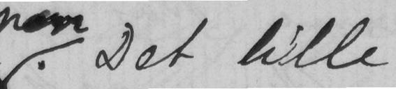. Det lille
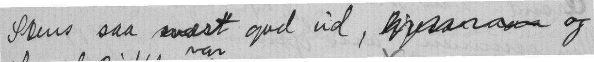Stens saa svært god ud,  og
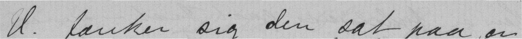V. tænker sig den sat paa en
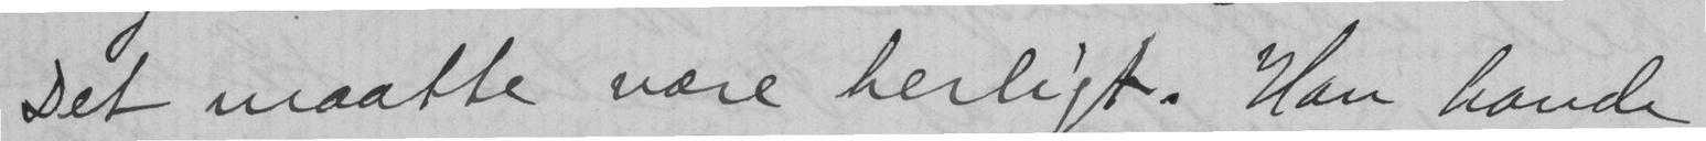Det maatte være herligt. Han havde
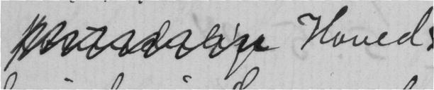Hoved
